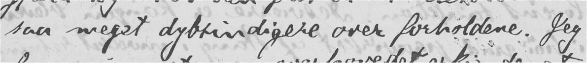saa meget dybsindigere over forholdene. Jeg
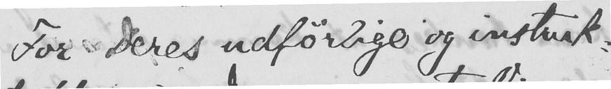For Deres udførlige og instruk-
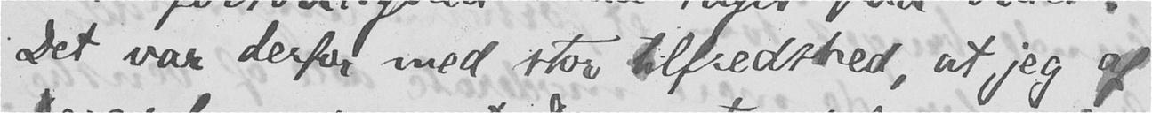Det var derfor med stor tilfredshed, at jeg af
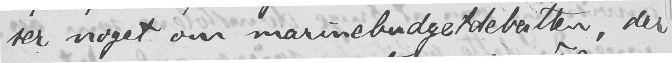ser noget om marinebudgetdebatten, der
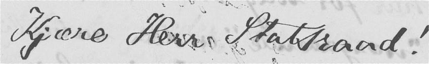Kjære Herr Statsraad!
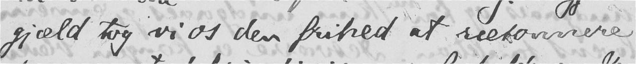gjæld tog vi os den frihed at ræsonnere
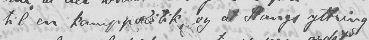til en kamppolitik, og at Stangs yttring
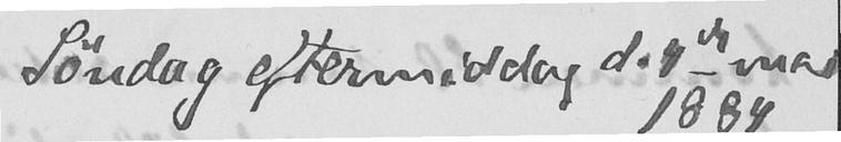Søndag eftermiddag d. 4de mai
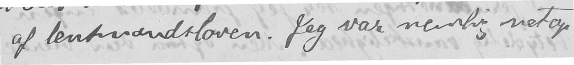af lensmandsloven. Jeg var nemlig netop
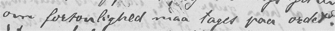om forsonlighed maa tages paa ordet.
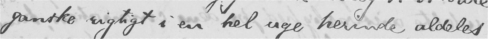ganske rigtigt i en hel uge herinde aldeles
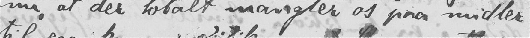nu, at der totalt mangler os paa midler
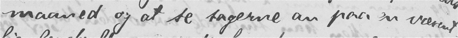maaned og at se sagerne an paa en væsent-
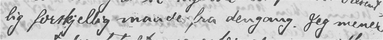lig forskjellig maade fra dengang. Jeg mener
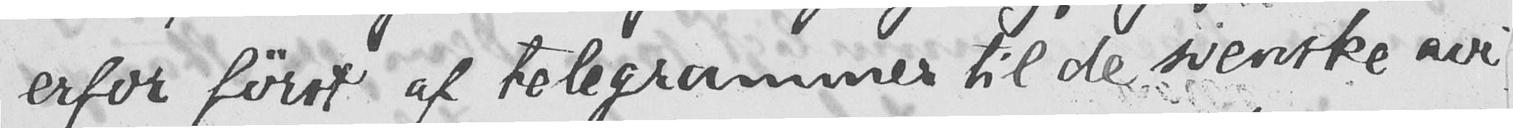erfor først af telegrammer til de svenske avi-
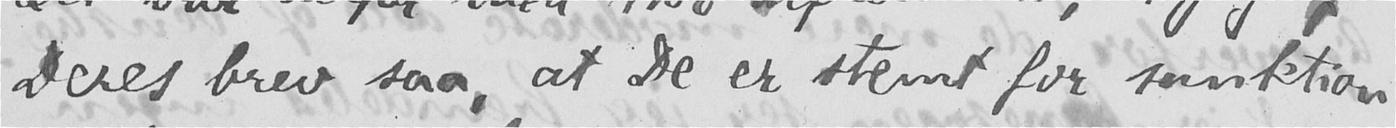Deres brev saa, at De er stemt for sanktion
